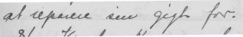at reparere sin gigt for.
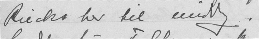Riecks her til middag.
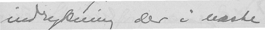indrykning der i næste
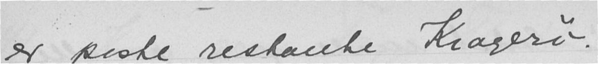er poste restante Kragerø.
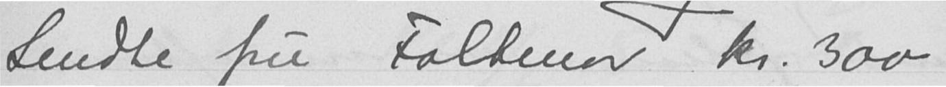Sendte fru Foltmar kr. 300
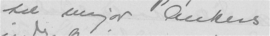til major Ankers
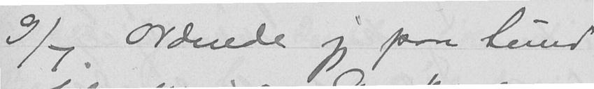9/7 Ordnede jeg paa Sund
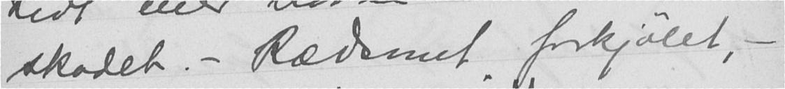skadet. - Rædsomt forkjølet, -
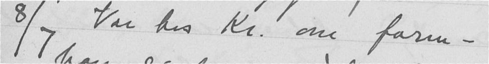8/7 Var hos Kr. om form -
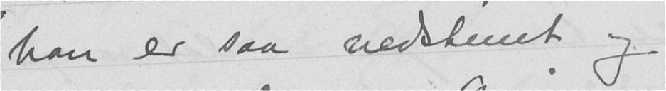han er saa nedstemt og
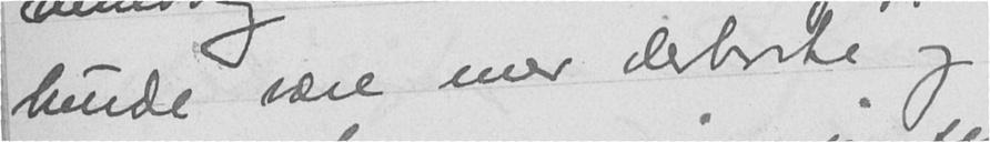burde være mer derborte og
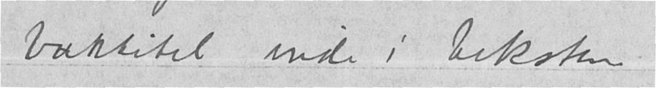boktitel inde i teksten.
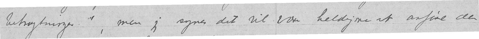betragtninger", men jeg synes det vil være heldigere at anføre den
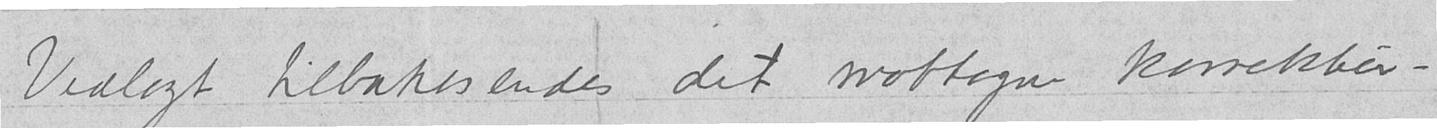Vedlagt tilbakesendes det mottagne korrektur-
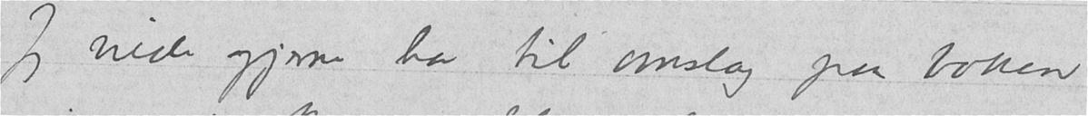Jeg vilde gjerne her til omslag paa boken
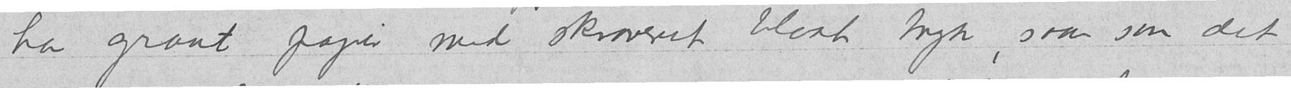ha graat papir med skraveret blaat tryk, saan som det
